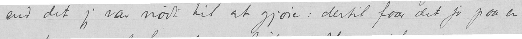end det jeg var nødt til at gjøre: dertil faar det jo paa en
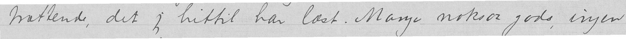trættende, det jeg hittil har læst. Mange noksaa gode, ingen
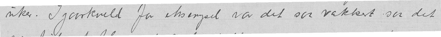uker. Igaarkveld for eksempel var det saa vakkert saa det
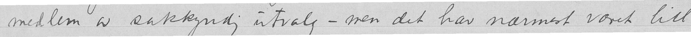medlem av sakkyndig utvalg – men det har nærmest været litt
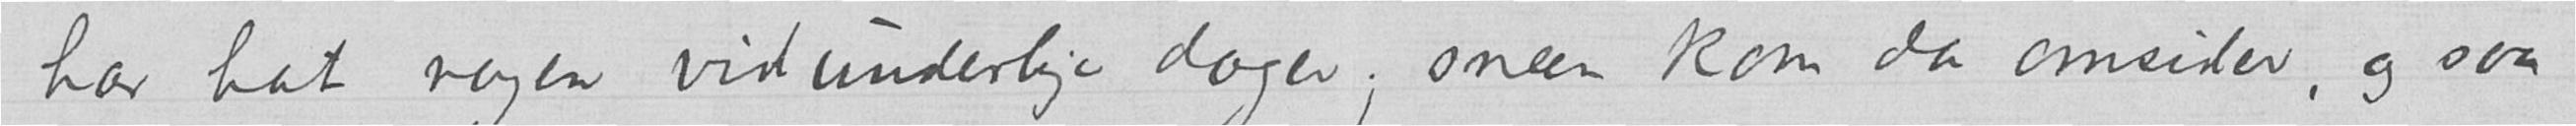har hat nogen vidunderlige dager; sneen kom da omsider, og saa
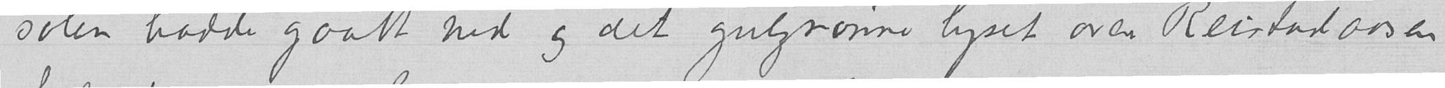solen hadde gaatt ned og det gulgrønne lyset over Reistadaasen
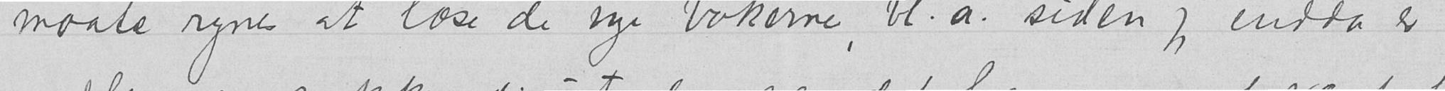maate regnes at læse de nye bøkerne, bl.a. siden jeg endda er
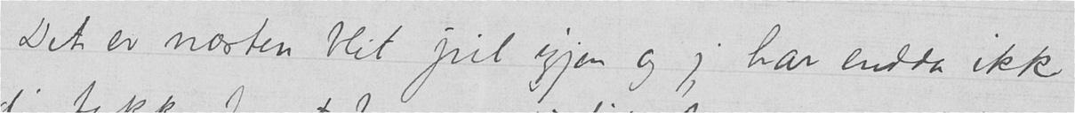Det er næsten blit jul igjen og jeg har endda ikke
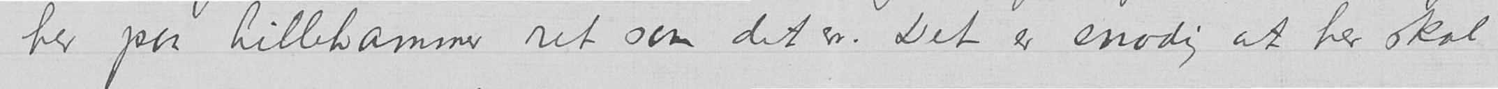her paa Lillehammer ret som det er. Det er snodig at her skal
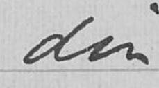din
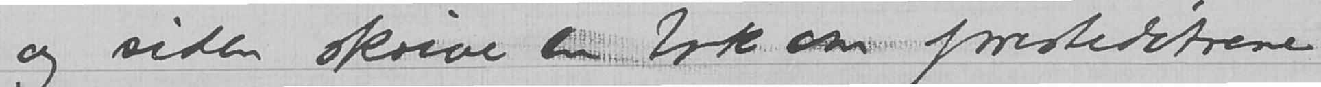og siden skrive en bok om prestedøtrene
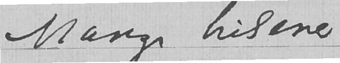Mange hilsener
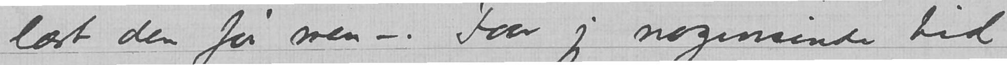læst dem før men .- Faar jeg nogensinde tid
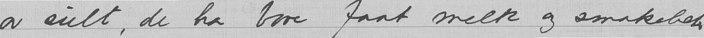av sult, de har bare faat melk og smakebeter
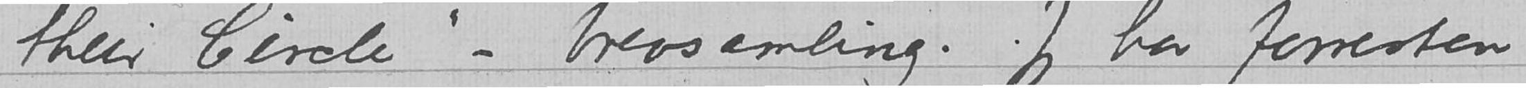their Circle» – brevsamling. Jeg har forresten
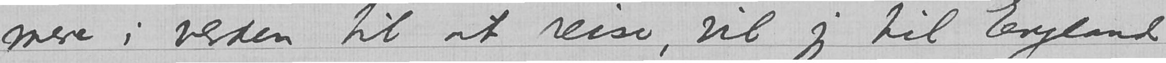mere i verden til at reise, vil jeg til England
